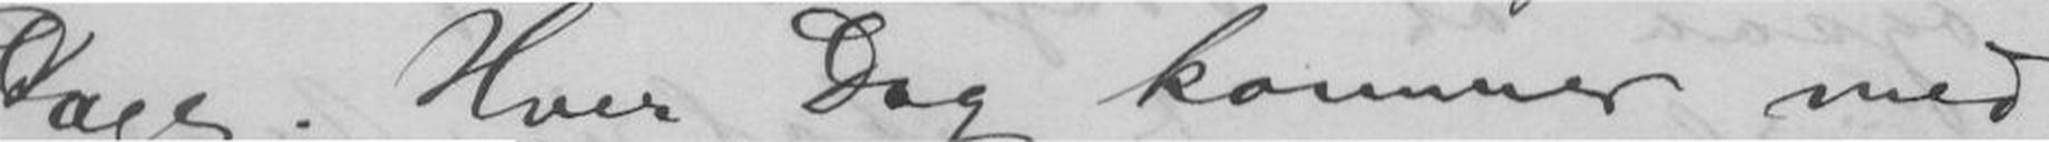Dage. Hver Dag kommer med
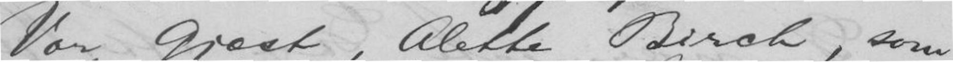Vor Gjæst, Alette Birch, som
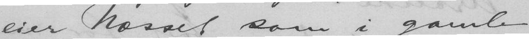eier Næsset som i gamle
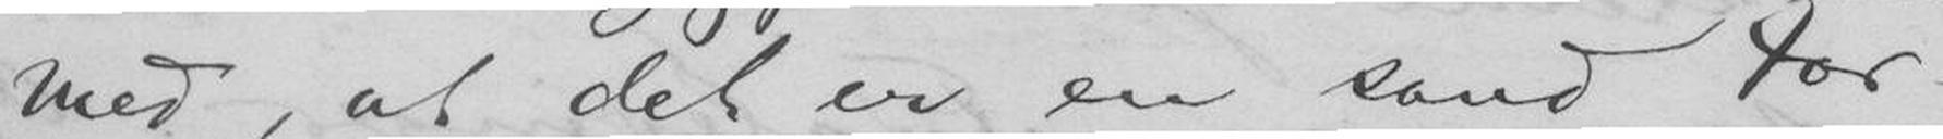med, at det er en sand For-
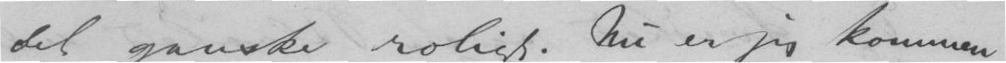det ganske roligt. Nu er jeg kommen
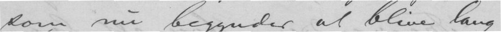som nu begynder at blive lang-
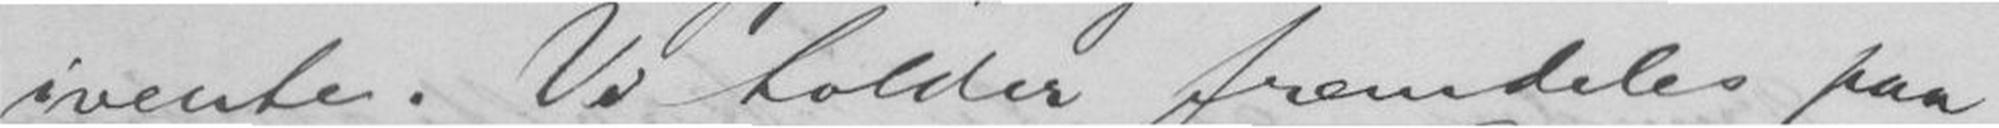ivente. Vi holder fremdeles paa
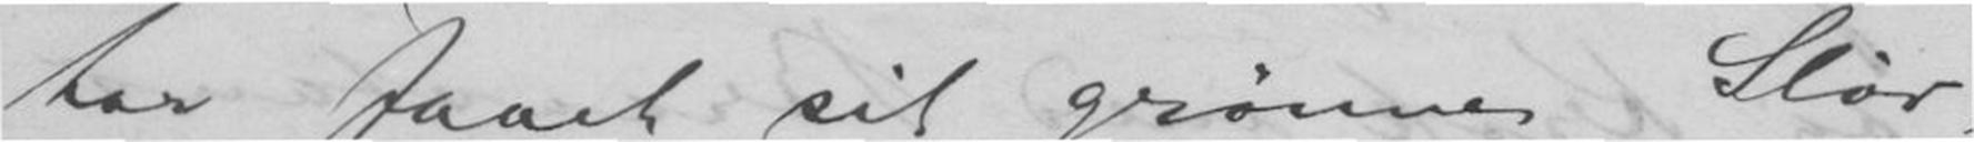har faaet sit grønne Slør,
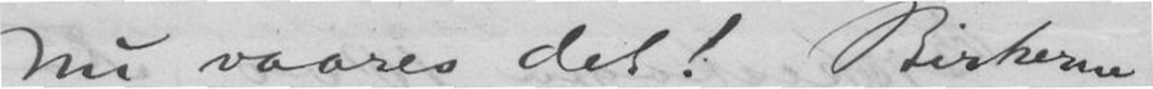Nu vaares det! Birkerne
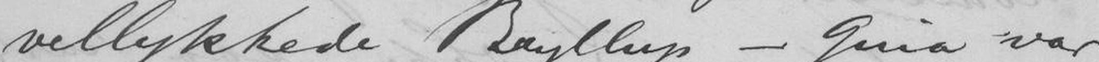vellykkede Bryllup - Gina var
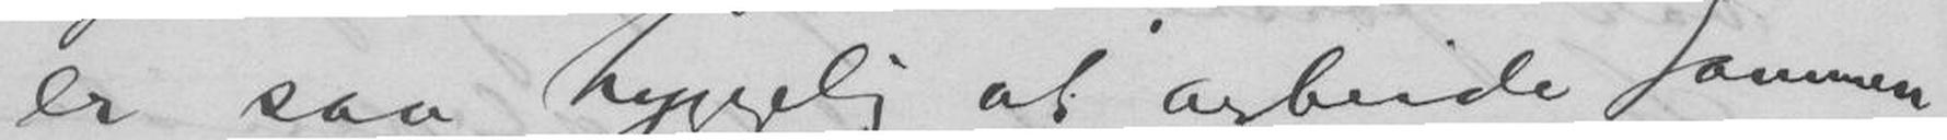er saa hyggelig at arbeide sammen
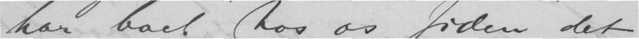har boet hos os siden det
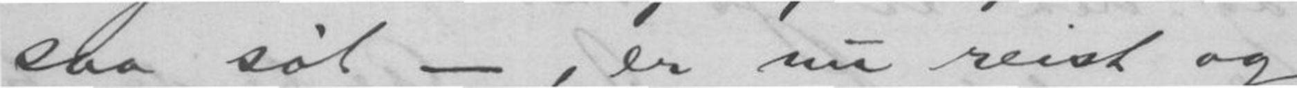saa sød -, er nu reist og
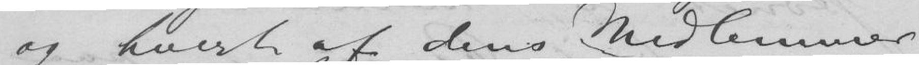og hvert af dens Medlemmer
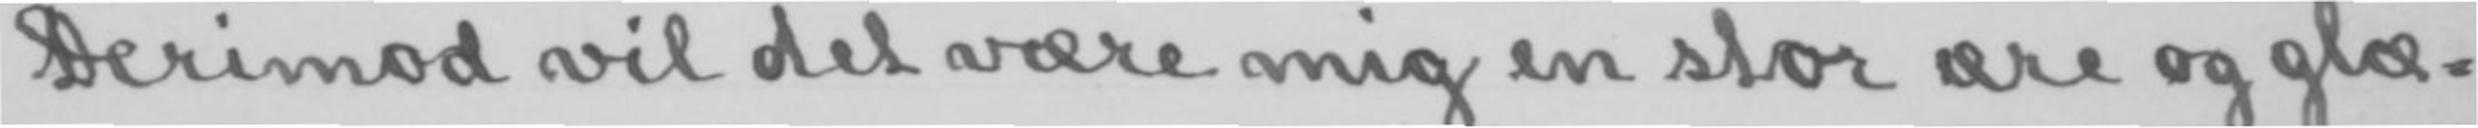Derimod vil det være mig en stor ære og glæ-
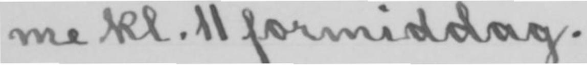me kl. 11 formiddag.
